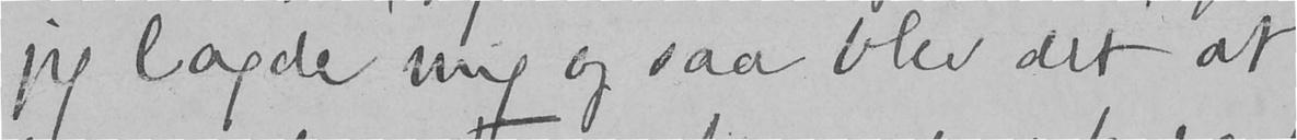jeg lagde mig og saa blev det at
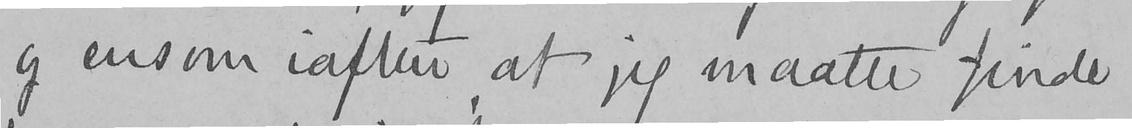og ensom iaften, at jeg maatte finde
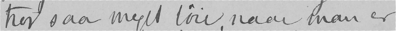tror saa meget løie, naar man er
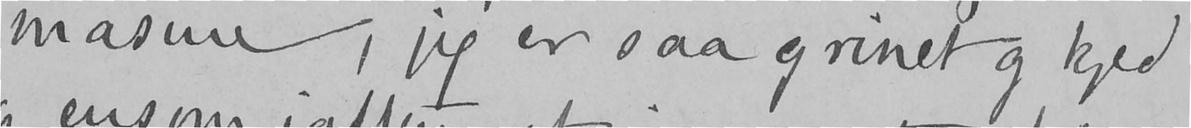masine, jeg er saa grinet og kjed
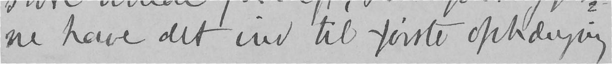ne have det ind til første ophænging
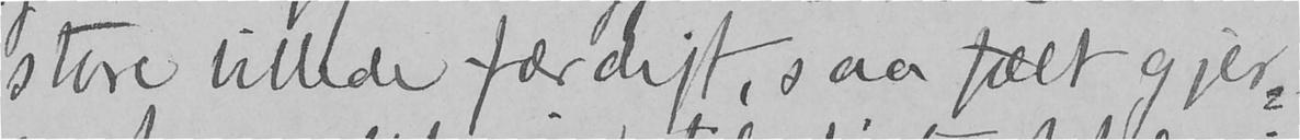store billede færdigt, saa fælt gjer-
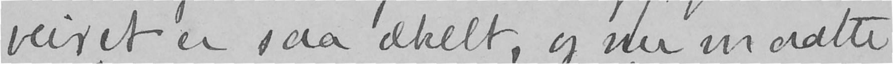veiret er saa ækelt, og nu maatte
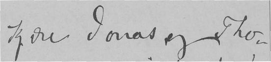Kjære Jonas og Tho-
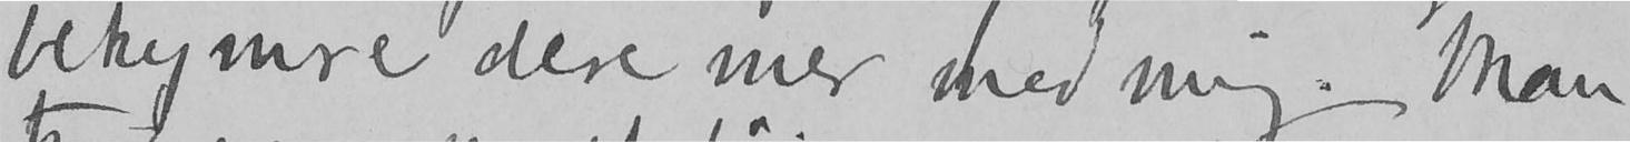bekymre dere mer med mig. Man
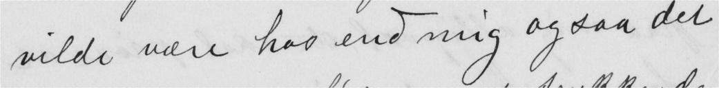vilde være hos end mig og saa det
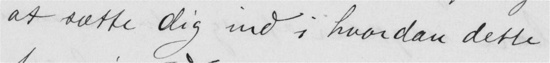at sætte dig ind i hvordan dette
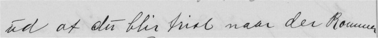ud at du blir trist naar der Kommer
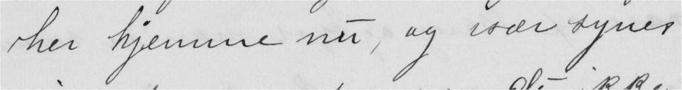her hjemme nu, og især synes
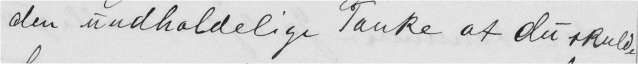den uudholdelige Tanke at du skulde
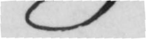3
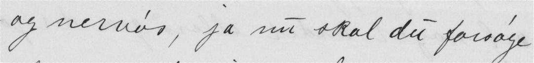og nervøs, ja nu skal du forsøge
